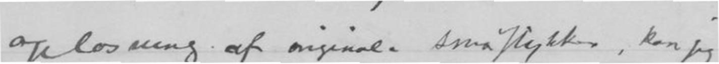oplæsning af original småstykker, kan jeg
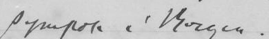sympati i Bergen.
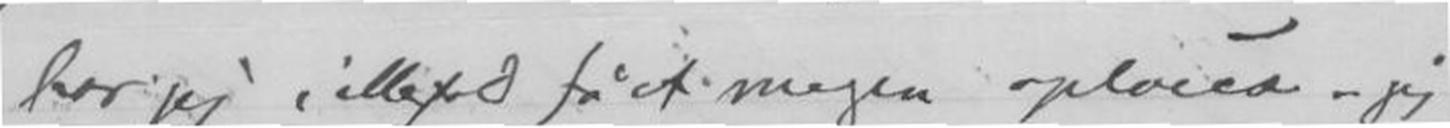har jeg iallefald fået megen aplaus - jeg
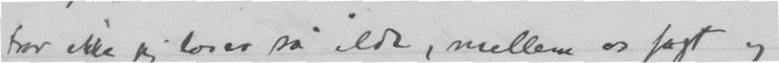tror ikke jeg læser så ilde, mellem os sagt og
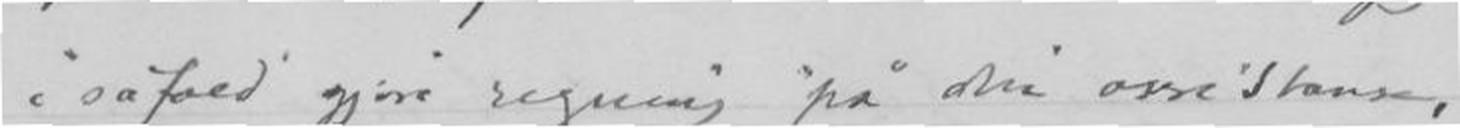i såfald gjøre regning på din assistanse,
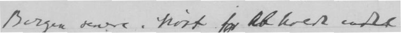Bergen senere i høst for det holdt indtet
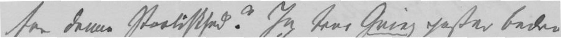for denne Partiskhed? Jeg tror Grieg passer bedre
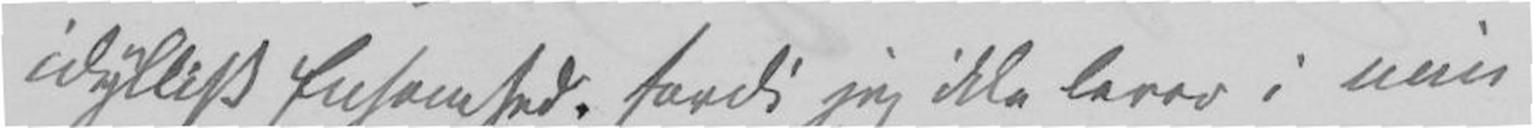idyllisk Ensomhed fordi jeg ikke lærer i min
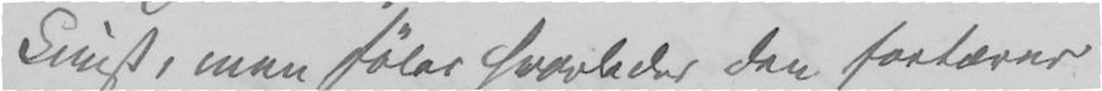Kunst, men føler hvorledes den fortærer
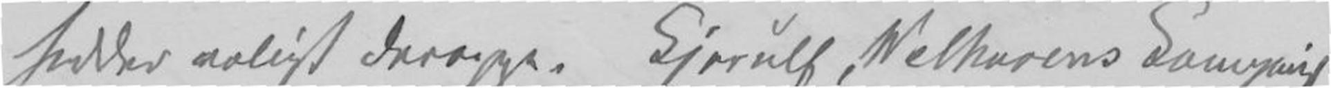sidder roligt deroppe. Kjerulf, Welhavens Komponist
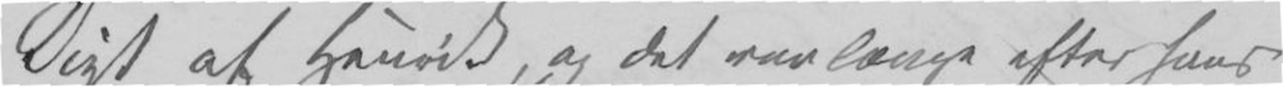Digt af Henrik, og det var længe efter hans
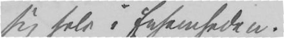sig selv i Ensomheden.
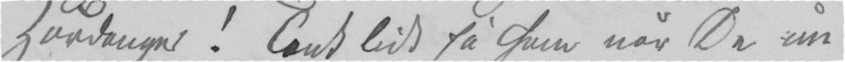Hardanger! Tænk lidt på ham når De nu
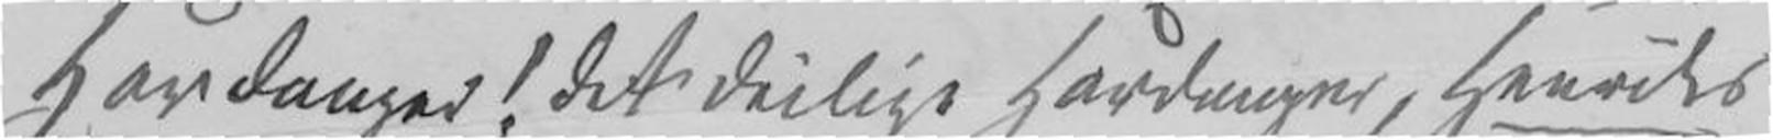Hardanger! Det deilige Hardanger, Henriks
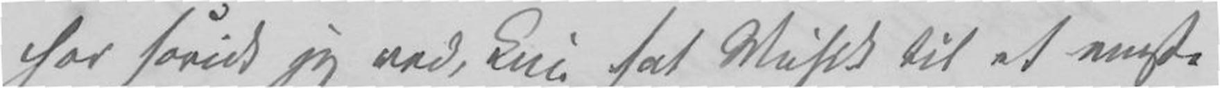har såvidt jeg ved, kun sat Musik til et eneste
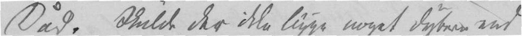Død. Skulde der ikke ligge noget dybere end
